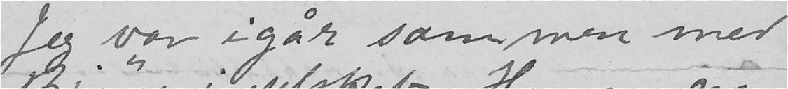Jeg var igår sammen med
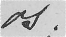os.
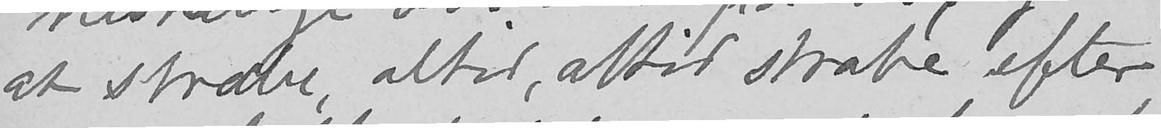at stræbe altid, altid stræbe efter
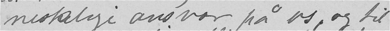neskelige ansvar på os, og til
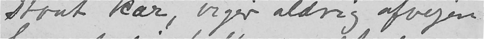stout kar, viger aldrig afvejen
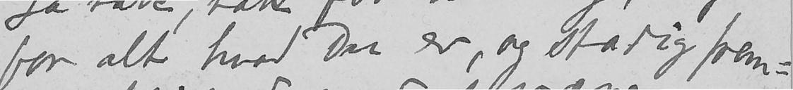for alt hvad Du er, og stadig frem-
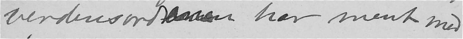verdensordenen har ment med
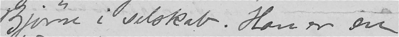Bjørn i selskab. Han er en
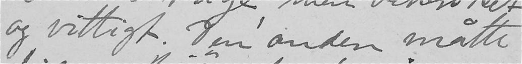og vittigt. Den anden måtte
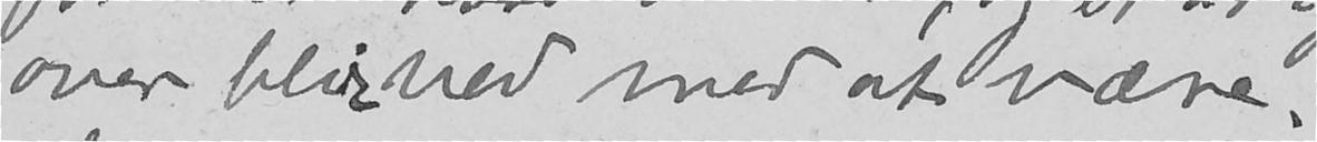over blir ved med at være.
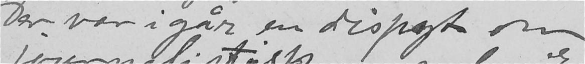Der var i går en dispyt om
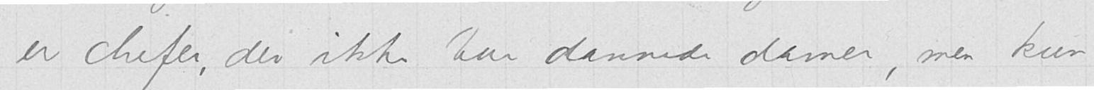er chefer, der ikke tar dannede damer, men kun
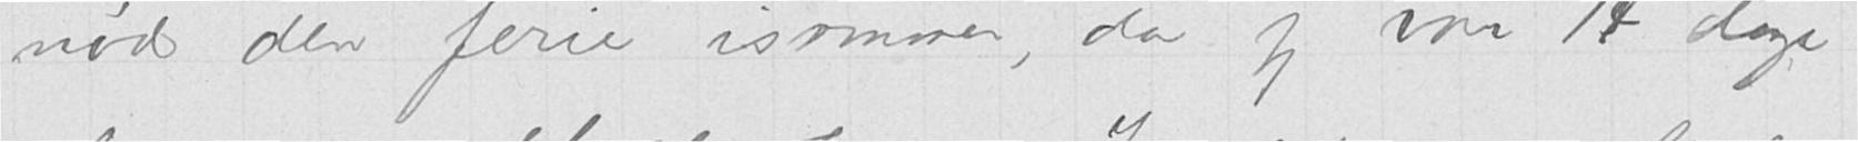nød den ferie isommer, da jeg var 14 dager
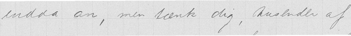endda an, men tænk dig, tusender af
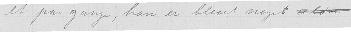et par gange, han er blevet noget ældre
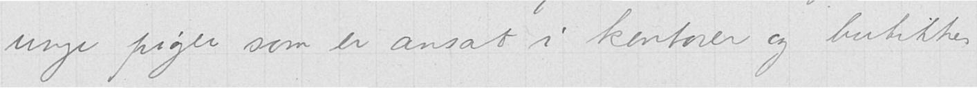unge piger som er ansat i kontorer og butikker,
